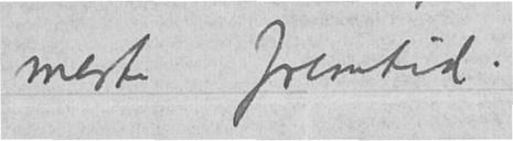meste fremtid.
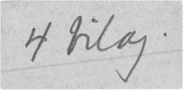4 bilag.
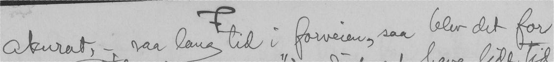akurat, - var lang tid i forveien, saa blev det for
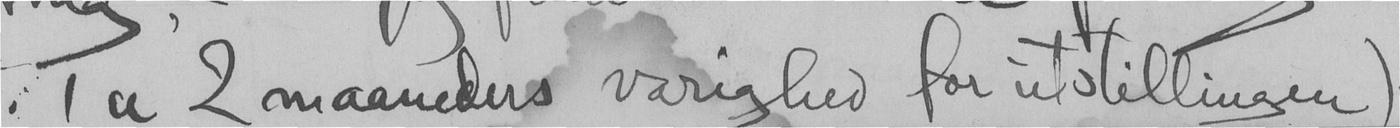i 1 a 12 maaneders varighed for ustillingen)
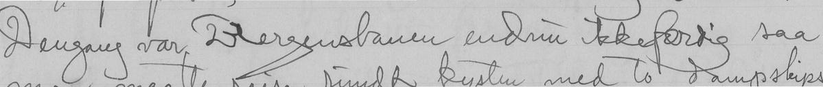Dengang var Bergensbanen endnu ikkefærdig saa
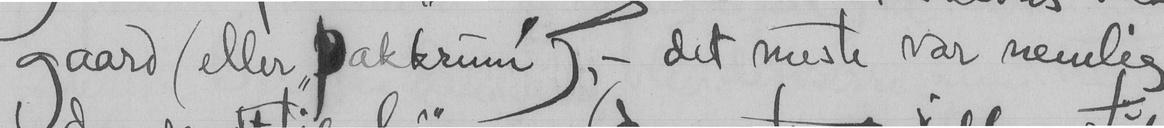gaard (eller "pakkrum"), - det meste var nemlig
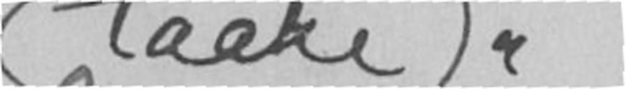(taake)
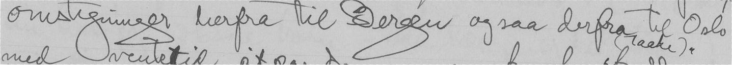omstigninger herfra til Bergen og saa derfra til Oslo
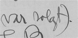var solgt).
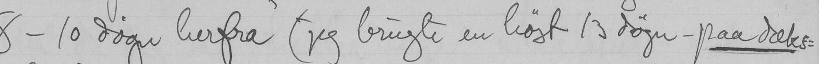8 - 10 dage herfra (jeg brugte en høst 13 døgn - paa dæks-
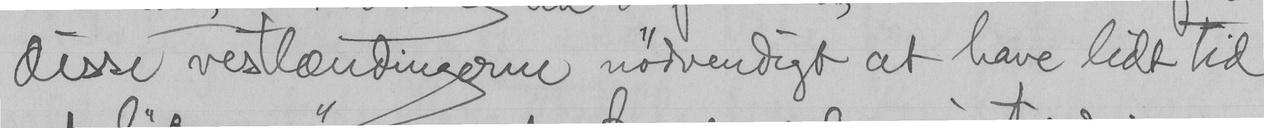disse vestlændingerne nødvendigt at have lidt tid
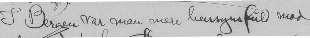I Bergen var man mere hensynsfuld mod
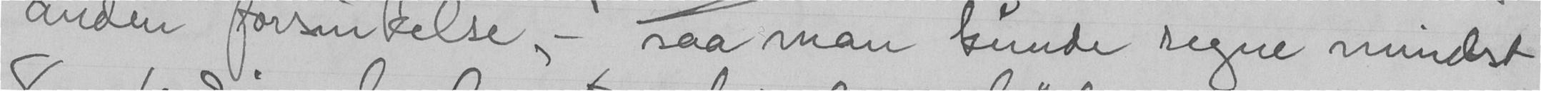anden forsinkelse, - saa man kunde regne mindst
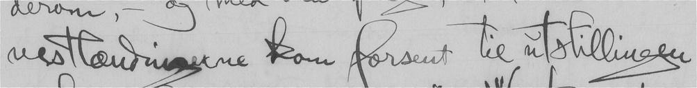vestlændingerne kom forsent til utstillingen
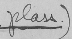plass)
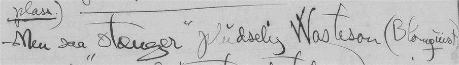Men saa "stænger"  pludselig Wasteson (Blomquist)
